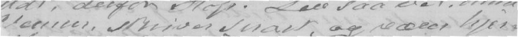Venner skriver snart, og væres hjer-
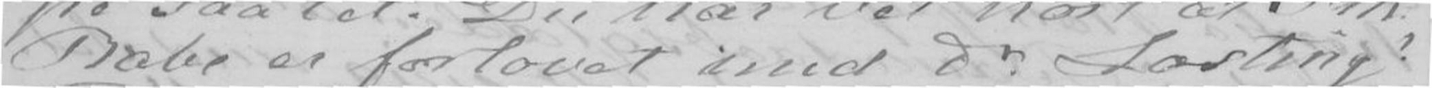Rabe er forlovet med Dr Losting?
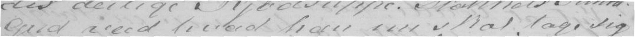Gud veed hvad han nu skal tage sig
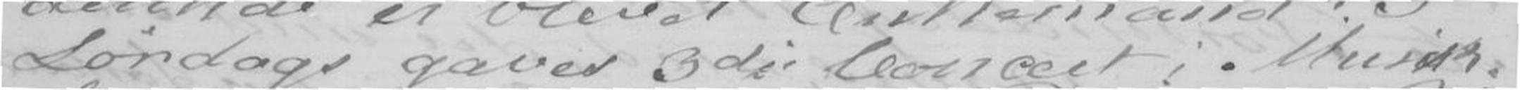Lørdags gaves 3die Concert i Musik-
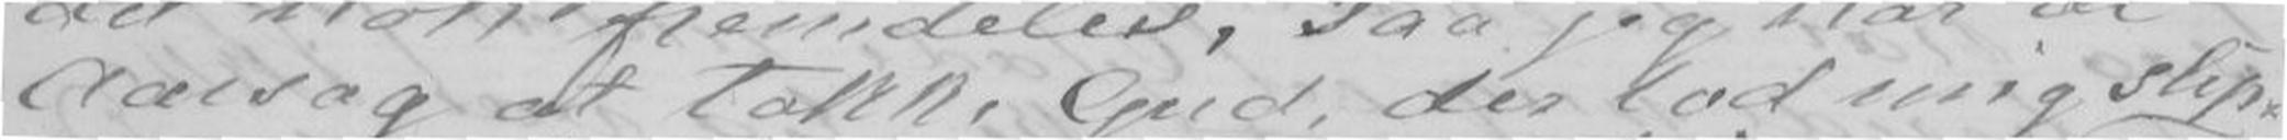Aarsag at takke Gud, der lod mig slip-
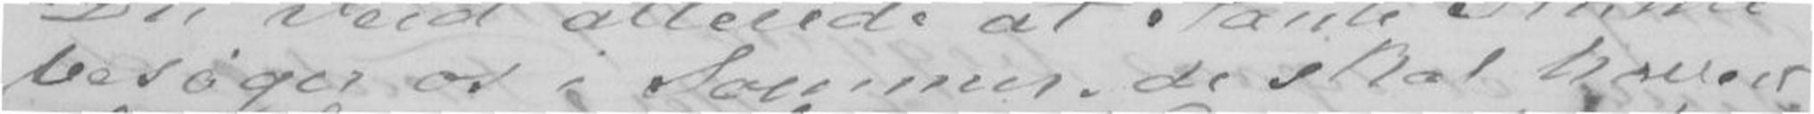besøger os i Sommer, de skal have et
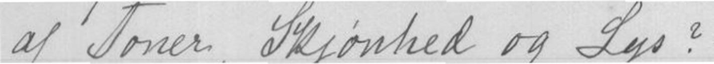af Toner, Skjønhed og Lys?
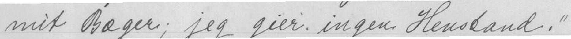mit Bæger; jeg gier ingen Henstand.
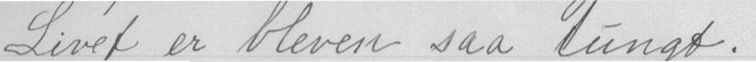Livet er bleven saa lungt.
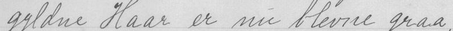gyldne Haar er nu blevne graa,
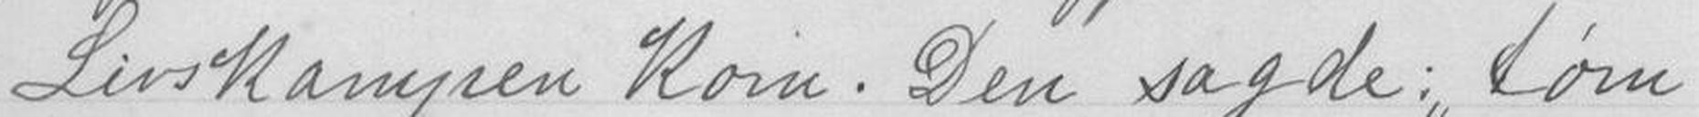Livskampen Kom. Den sagde: tøm
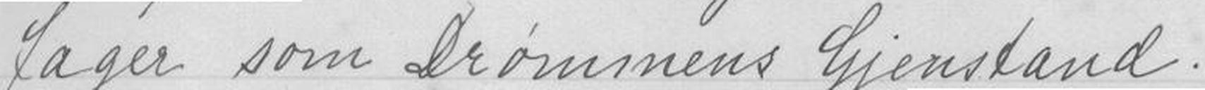fager som Drømmens Gjenstand
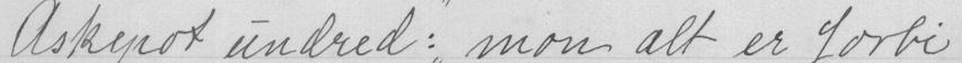Askepot undred: mon alt er forbi
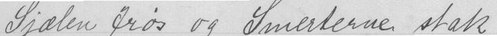Sjælen frøs og Smerterne stak -
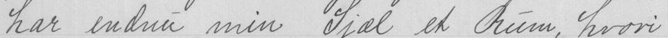har endnu min Sjæl et Rum, hvori
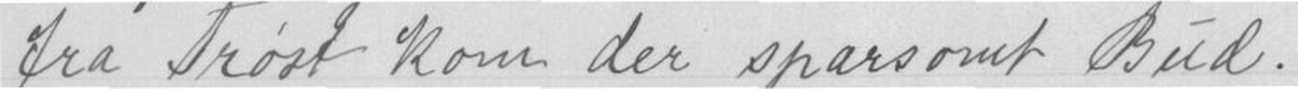fra Trøst kom der sparsomt Bud.
